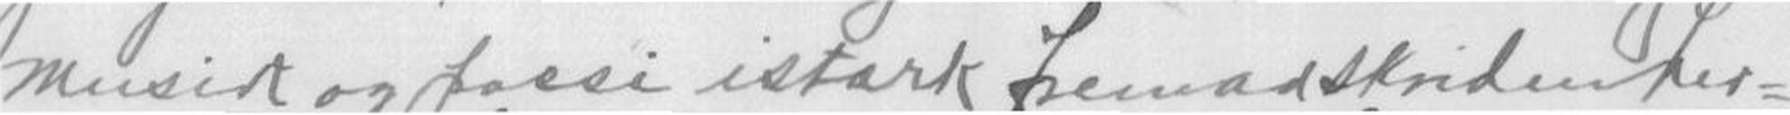Musik og poesi istærk fremadskriden her-
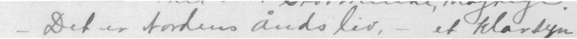- -Det er tordens Ånds liv, et klarsyn,
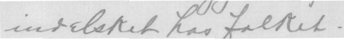indelsket hos folket.
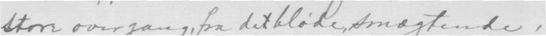store overgang, fra de bløde, smægtende,
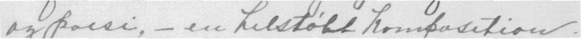og poesi, - en helstøbt komposition.
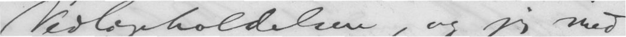Vedligeholdelsen, og jeg med
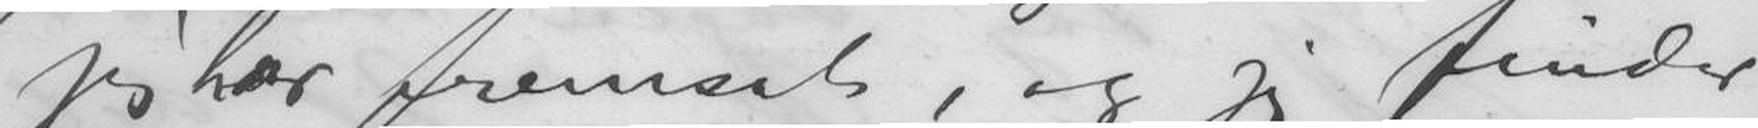jeg har fremsat, og jeg finder
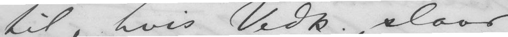til, hvis Vedk. slaar
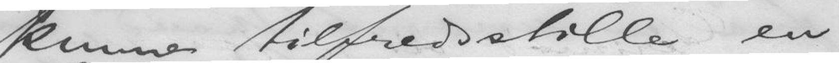kunne tilfredstille en
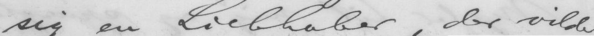sig en Liebhaber, der vilde
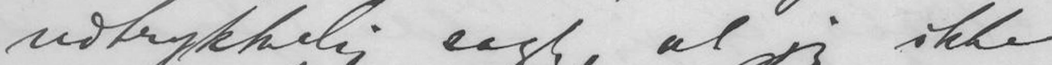udtrykkelig sagt, at jeg ikke
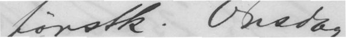førstk. Onsdag
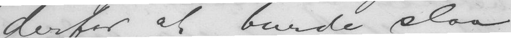derfor at burde slaa
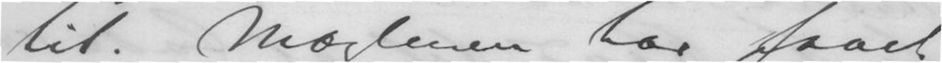til. Mægleren har faaet
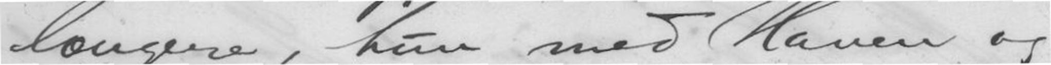længere, hun med Haven og
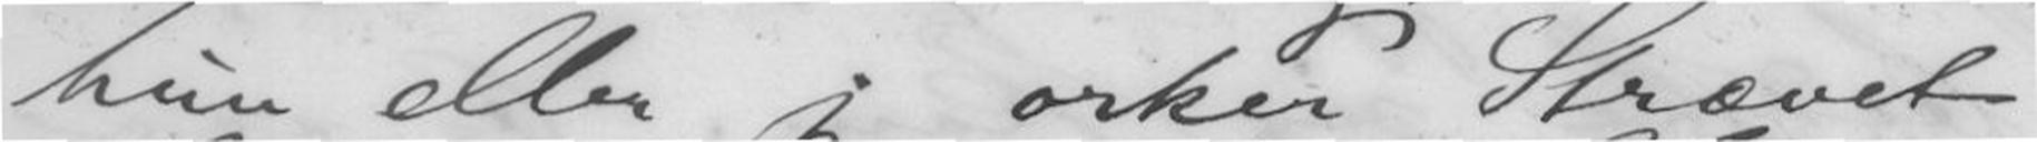hun eller jeg orker Strævet
